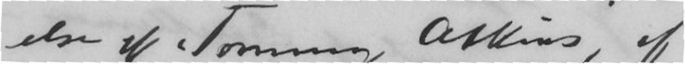else af, Tommy Atkins, af
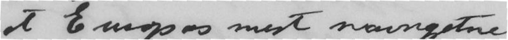at Europas mest navngjetne
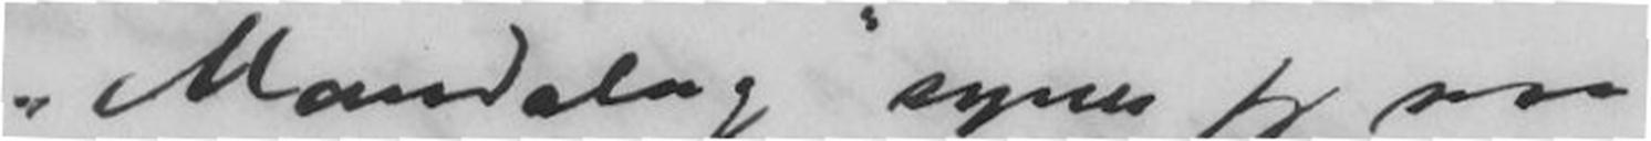Mandalen synes jeg saa
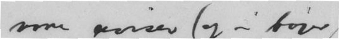som aviser (og i bøv)
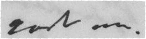godt om.
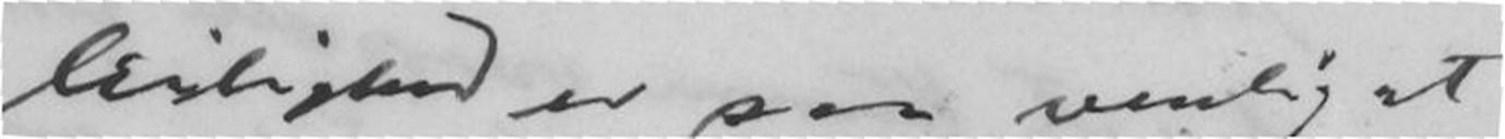Leilighed er saa venlig at
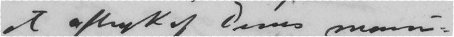et aftryk af Deres manu-
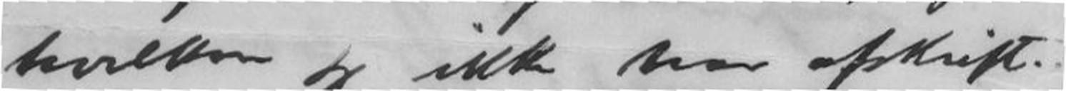hvilken jeg ikke afskrift
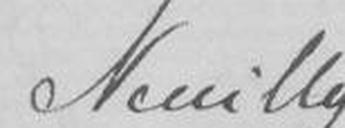Neuilly
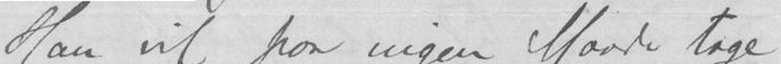Han vil for ingen Mande tage
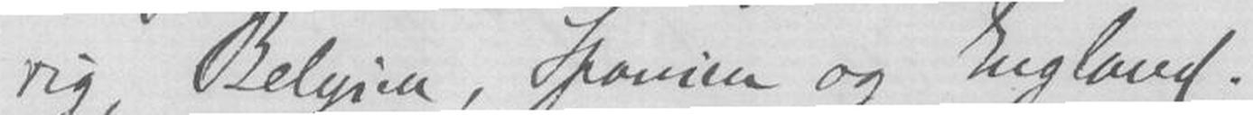rig, Belgia, Spania og England.
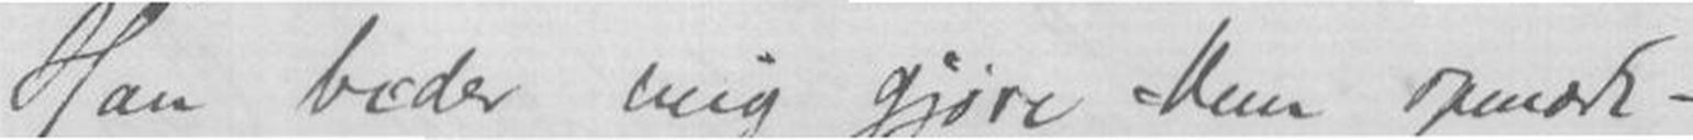Han beder mig gjøre Dem opmærk-
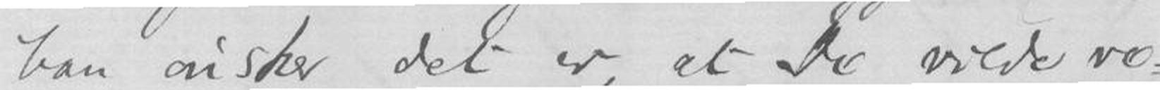han ønsker det er, at De vilde re-
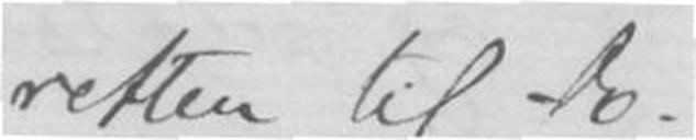retten til De-
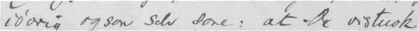iøvrig ogsaa selv sore: at De vistnok
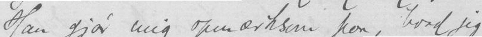Han gjør mig opmærksom paa, hvad jeg
